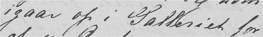i gaar op i Galleriet for
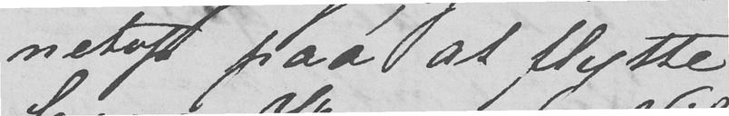netop paa at flytte
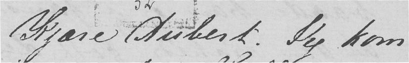Kjære Aubert. Jeg kom
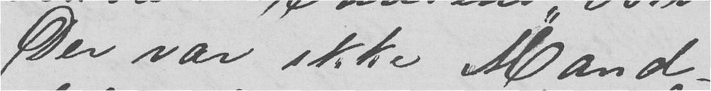Der var ikke Mand-
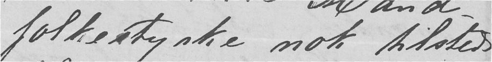folkestyrke nok tilstede
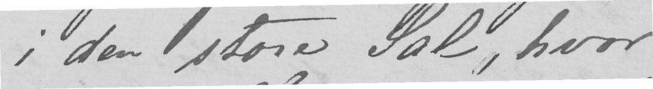i den store Sal, hvor
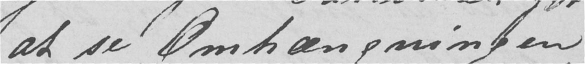at se Omhængningen
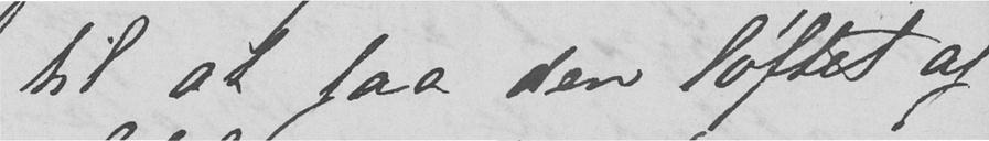til at faa den løftet af
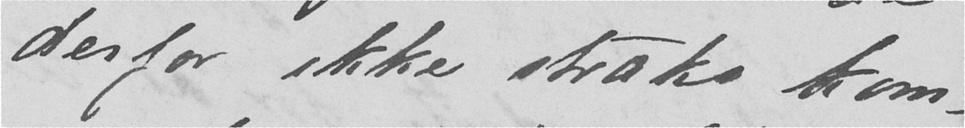derfor ikke straks kom-
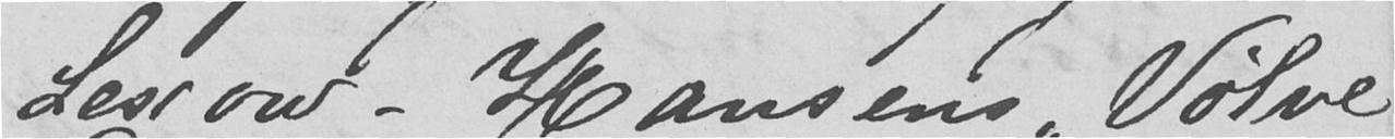Lexow-Hansens "Volve".
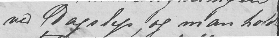ved Dagslys, og man holdt
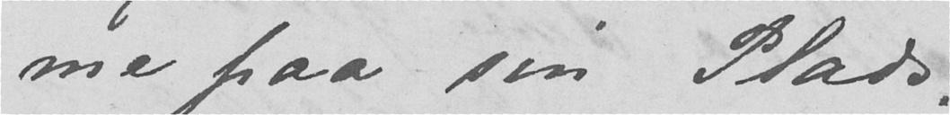me paa sin Plads.
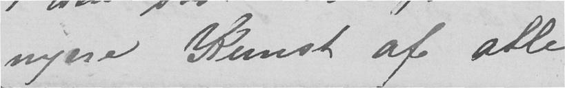nyere Kunst af alle
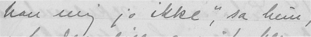han mig jo ikke", sa hun,
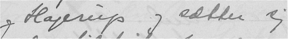og Hagerup og sætter sig
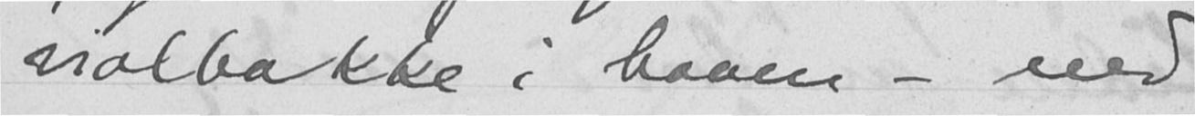violbakke i haven - ned
